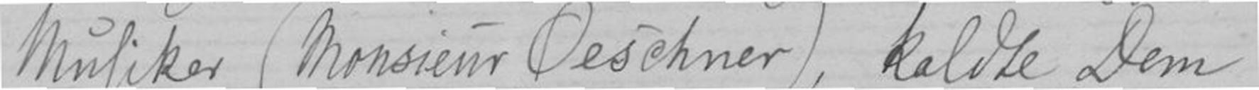Musiker (Monsieur Oeschner), kaldte Dem
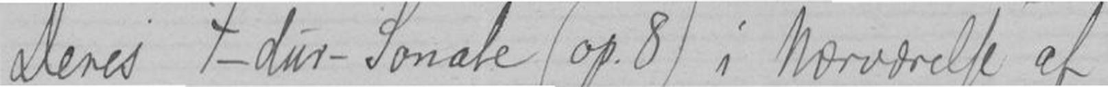Deres F-dur-Sonate (op 8) i Nærværlse af
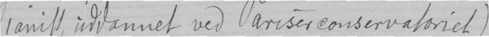Pianist, uddannet ved Pariserconservatoriet)
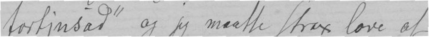"fortjusad og jeg maatte strax love at
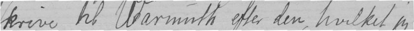skrive til Warmuth efter den, hvilket jeg
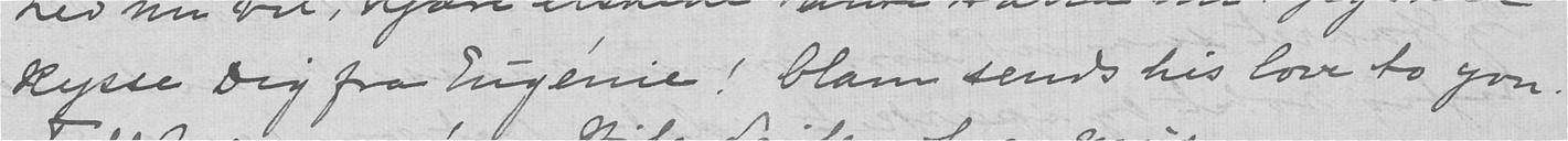Kysse Dig fra Eugénie! Olam sends his love to you.
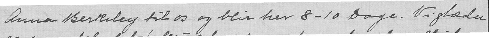Anna Berkeley til os og blir her 8-10 Dage. Vi glæder
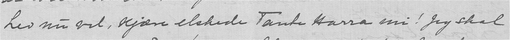Lev nu vel, kjære elskede Tante Harra mi! Jeg skal
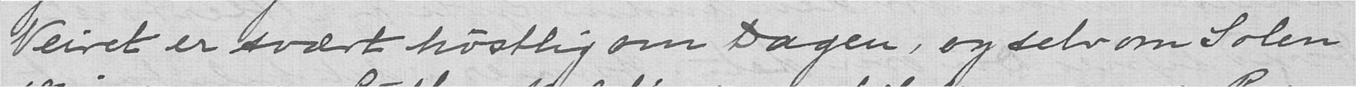Veiret er svært høstlig om Dagen, og selv om Solen
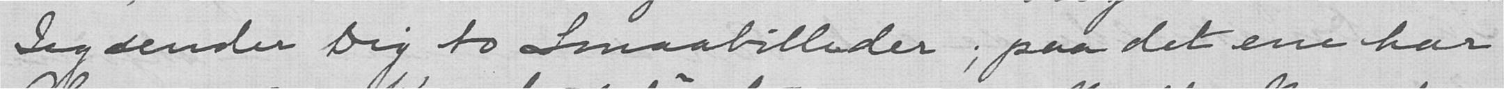Jeg sender Dig to Smaabilleder; paa det ene har
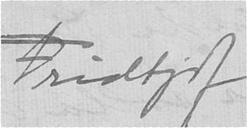Fridtjof
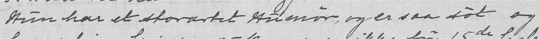Hun har et storartet Humør, og er saa søt og
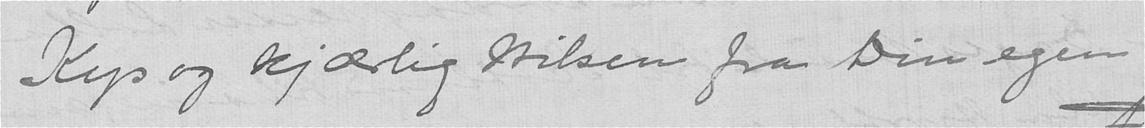Kys og kjærlig Hilsen fra Din egen
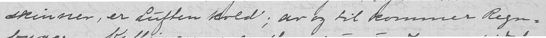skinner, er Luften kold; av og til kommer Regn-
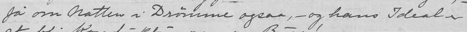Ja om Natten i Drømme ogsaa, - og hans Ideal er
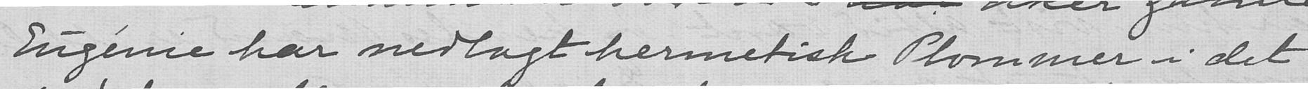Eugénie har nedlagt hermetisk Plommer i det
